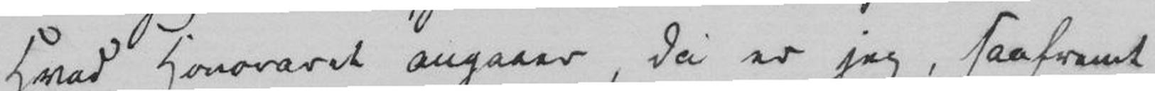Hvad Honoraret angaar, da er jeg, saafremt
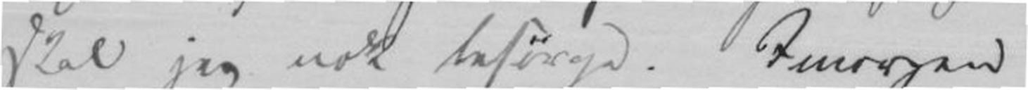skal jeg nok besørge. Imorgen
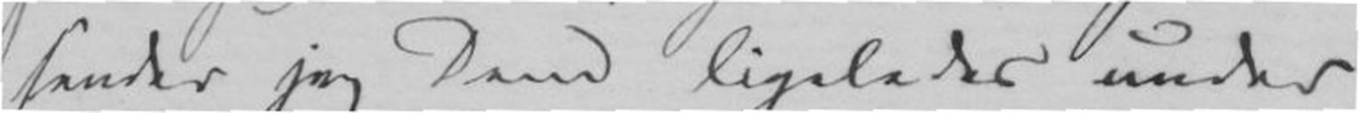sender jeg Dem ligeledes under
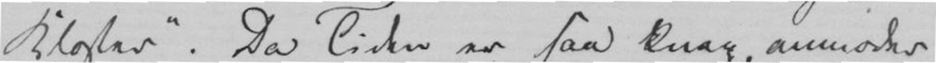Kloster". Da Tiden er saa knap, anmoder
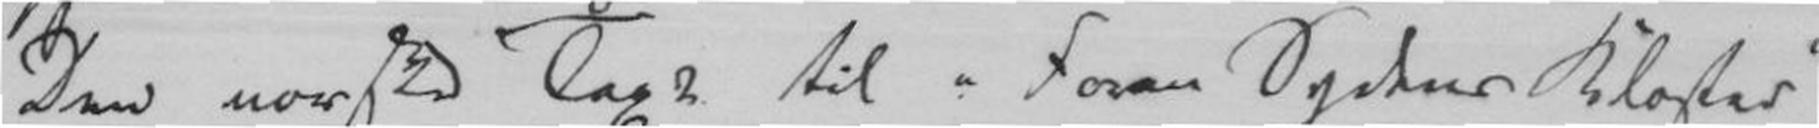Den norske Text til Foran Sydens Kloster
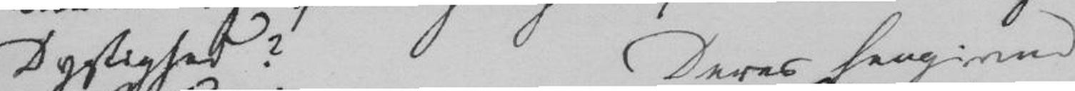Dygtighed?
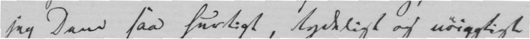jeg Dem saa hurtigt, tydeligt og nøiagtig,
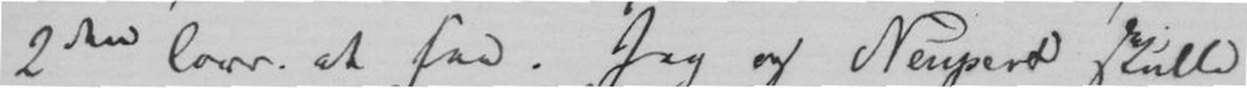2den Corr. at see. Jeg og Neupert skulle
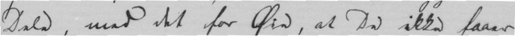Dele, med det for Øie, at De ikke faaer
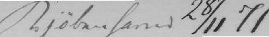Kjøbenhavn 28/11 71
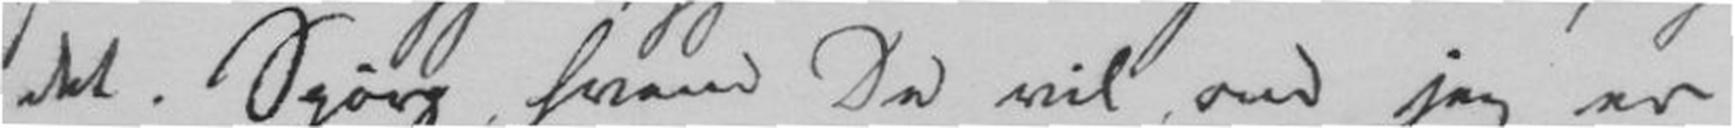det. Spørg hvem De vil om jeg er
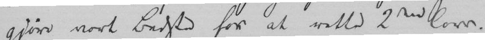gjøre vort Bedste for at rette 2den Corr.
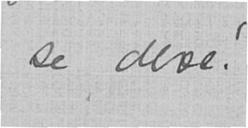se dere!
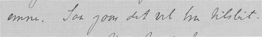emne. Saa gaar det vel bra tilslut.
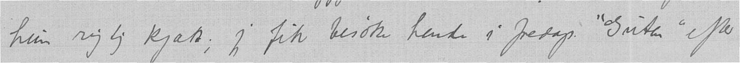hun rigtig kjæk; jeg fik besøke hende i fredags. "Guten" efter
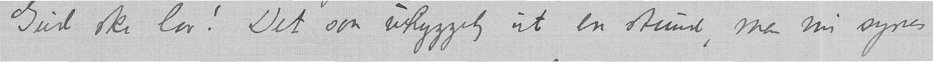Gud ske lov! Det saa uhyggelig ut en stund, men nu synes
